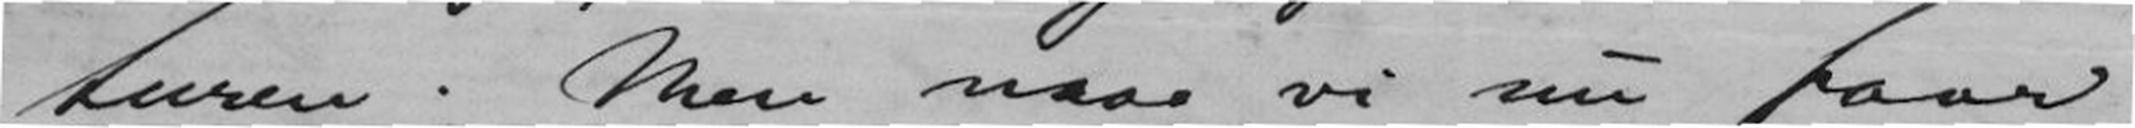turen. Men naar vi nu faar
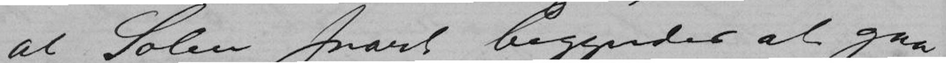at Solen snart begynder at gaa
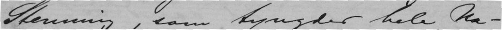Stemning, som tyngder hele Na-
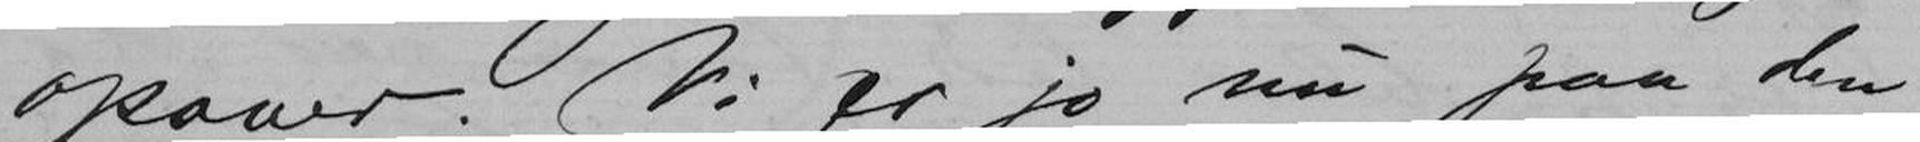opover. Vi er jo nu paa den
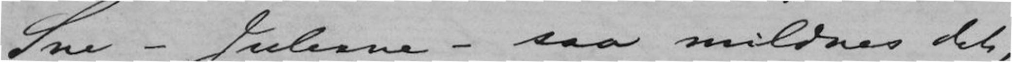Sne - Julesne - saa mildnes det,
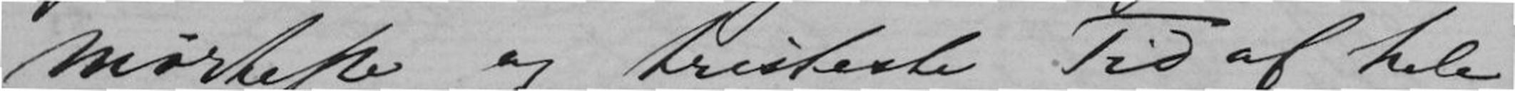Mørkeste og tristeste Tid af hele
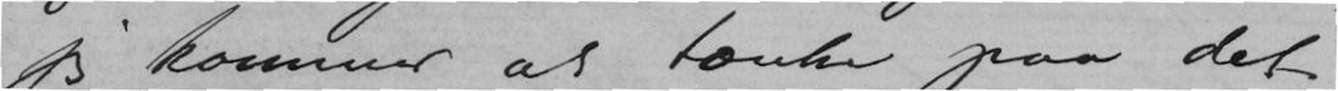jeg kommer at tænke paa det
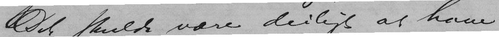Det skulde være deiligt at have
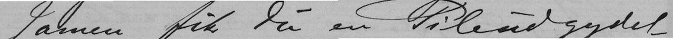Jamen fik du en Pileudgydel-
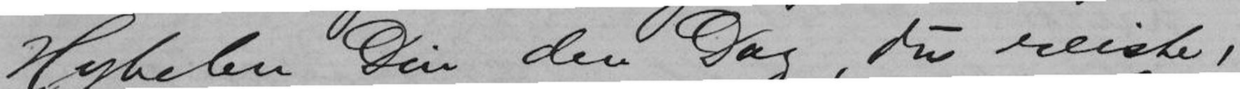Hybelen Din den Dag, du reiste!
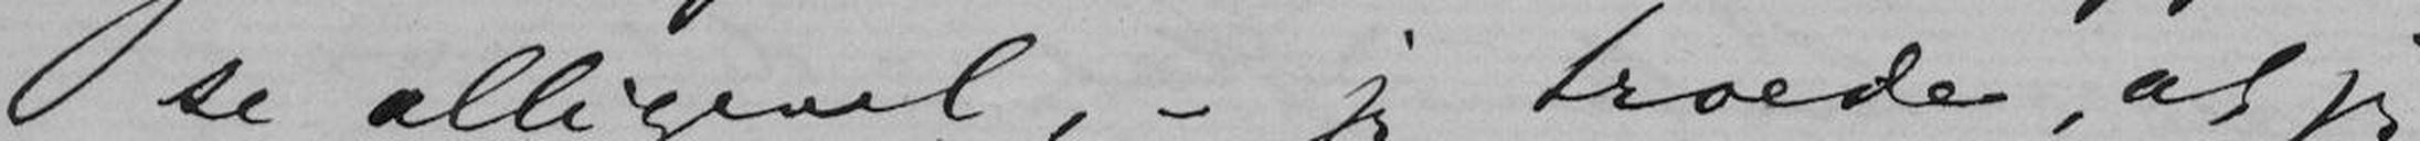se alligevel, - jeg troede, at jeg
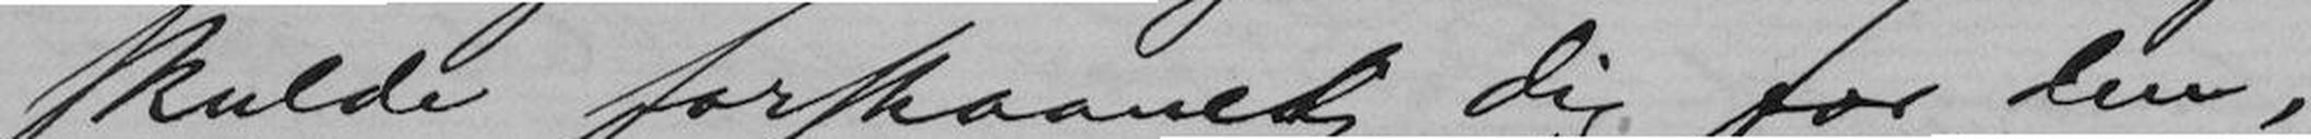skulde forskaanet dig for den,
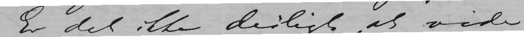- Er det ikke deiligt, at vide
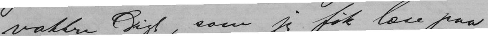vakkre Digt, som jeg fik læse paa
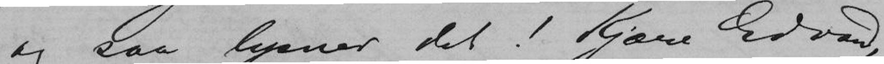og saa lysner det! Kjære Edvard,
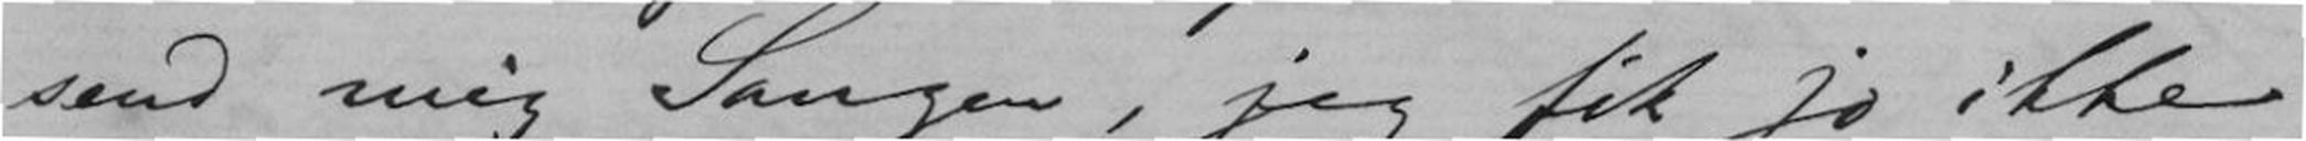send mig Sangen, jeg fik jo ikke
